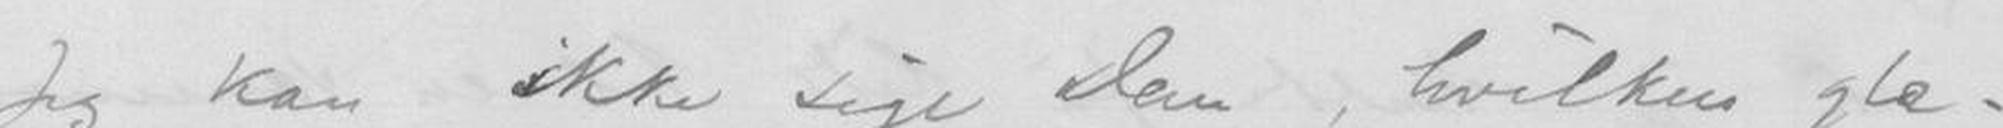Jeg kan ikke sige Dem, hvilken glæ-
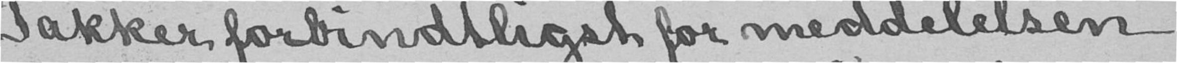Takker forbindtligst for meddelelsen.
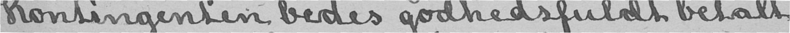Kontingenten bedes godhedsfuldt betalt
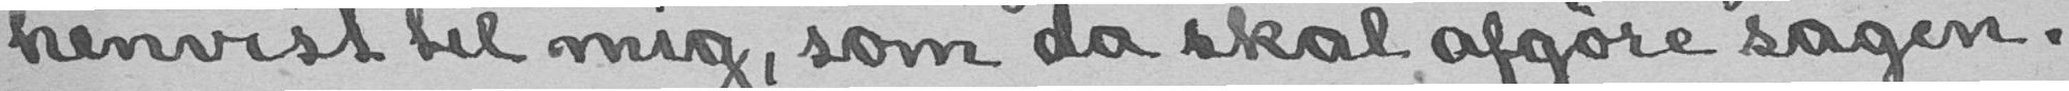henvist til mig, som da skal afgøre sagen.
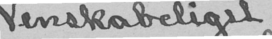Venskabeligst
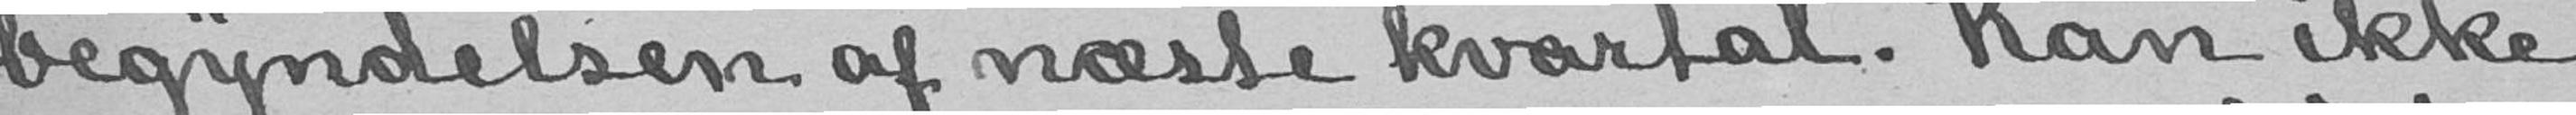begyndelsen af næste kvartal. Kan ikke
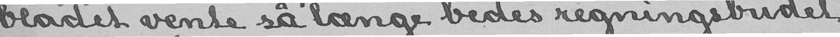bladet vente så længe bedes regningsbudet
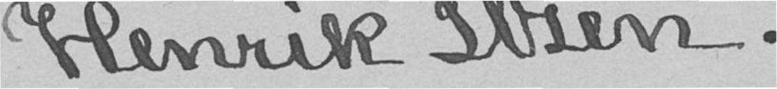Henrik Ibsen.
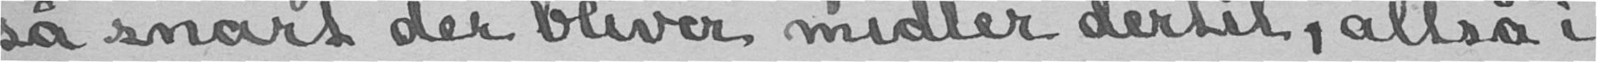så snart der bliver midler dertil, altså i
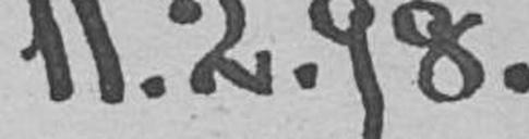11.2.98.
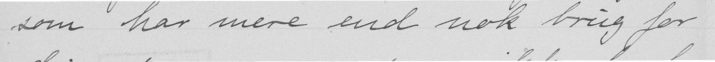som har mere end nok brug for
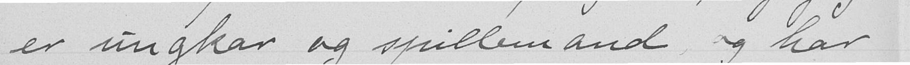er ungkar og spillemand, og har
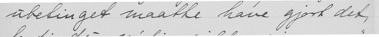ubetinget maatte have gjort det,
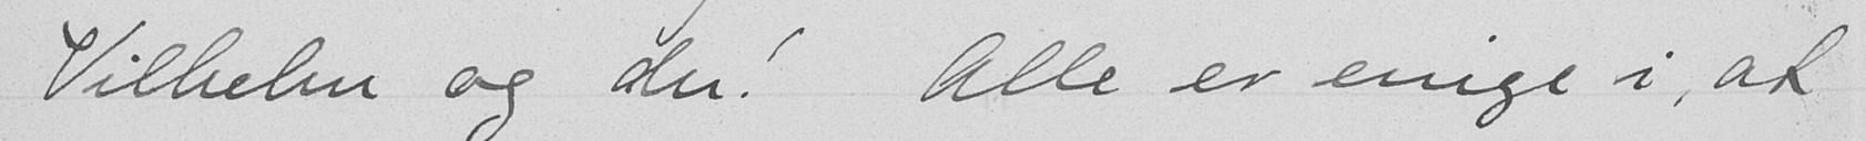Vilhelm og du! Alle er enige i, at
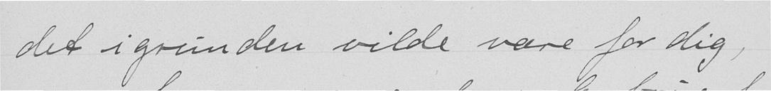det igrunden vilde være for dig,
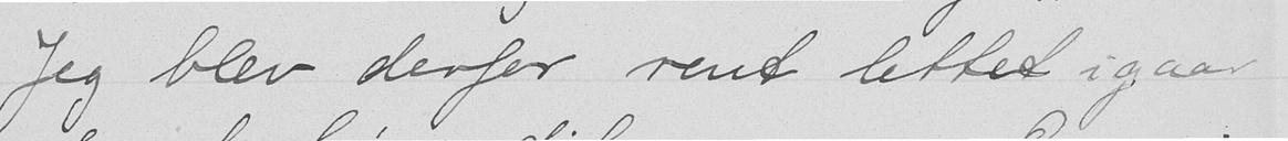Jeg blev derfor rent lettet igaar
